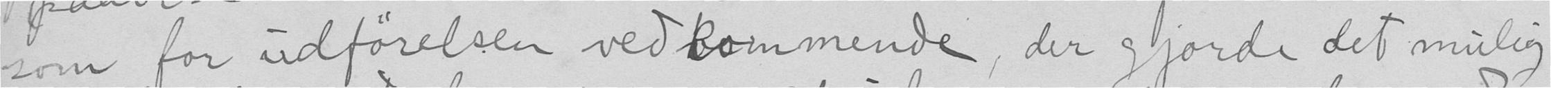som for udførelsen vedkommende, der gjorde det mulig
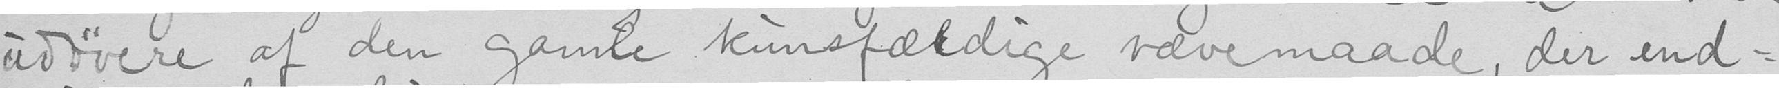udøvere af den gamle kunsfældige vævemaade, der end-
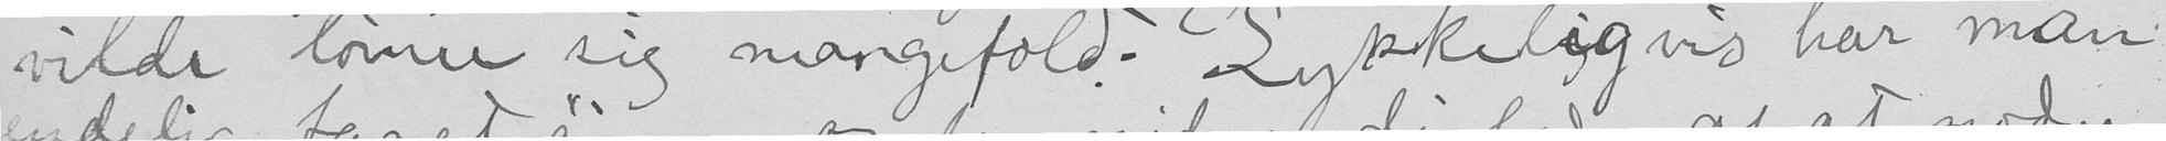vilde lønne sig mangefold. Lykkeligvis har man
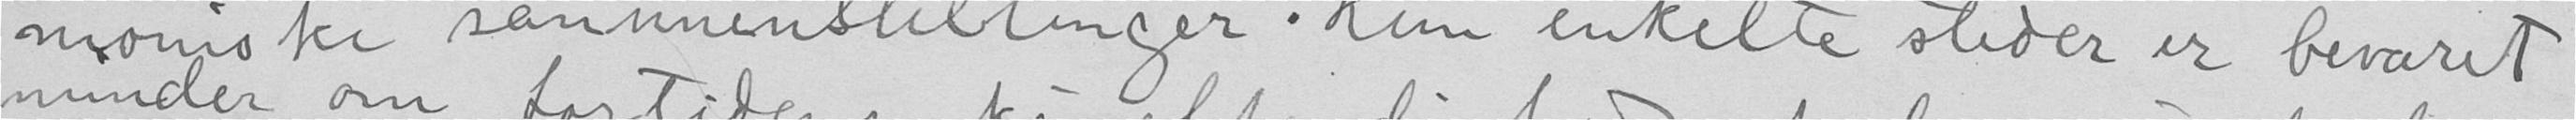moniske sammenstillinger. Kun enkelte steder er bevaret
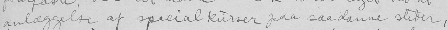anlæggelse af specialkurser paa saadanne steder,
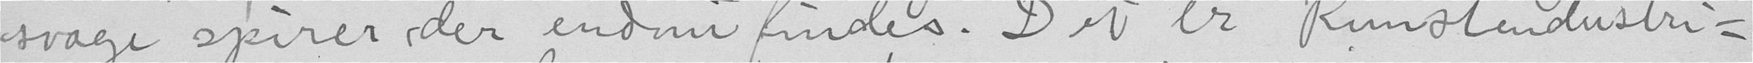svage spirer der endnu findes. Det er Kunstindustri-
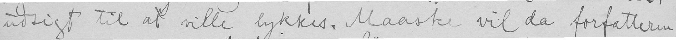udsigt til at ville lykkes, Maaske vil da forfatteren
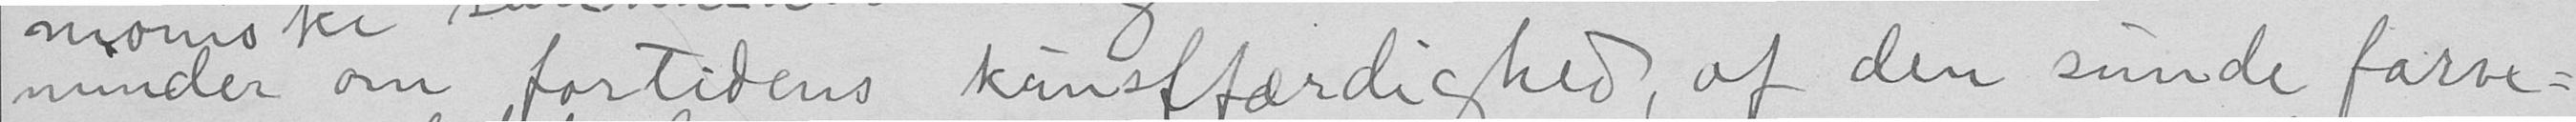minder om fortidens kunstfærdighed, af den sunde farve-
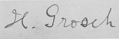H. Grosch
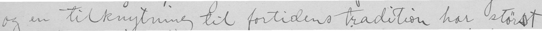og en tilknytning til fortidens tradition har størst
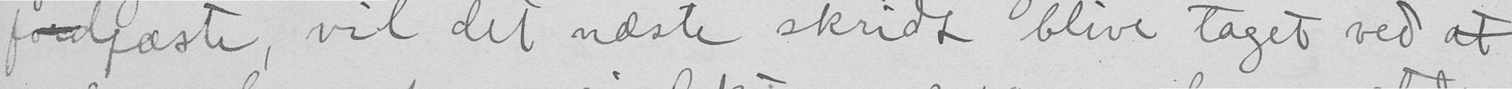fodfæste, vil det næste skridt blive taget ved at
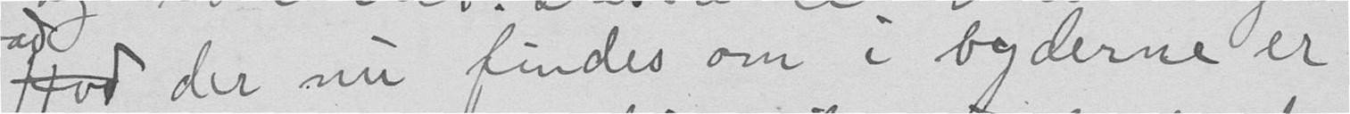du nu findes om i byderne er
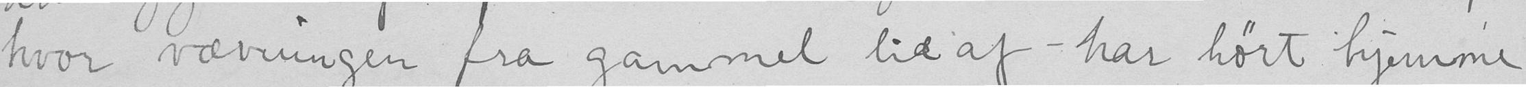hvor vævningen fra gammel tid af har hørt hjemme
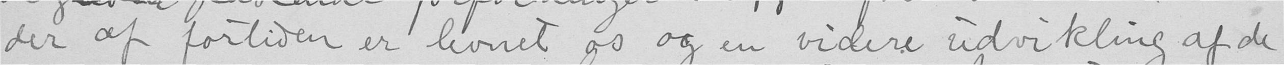der af fortiden er levnet os og en videre udvikling af de
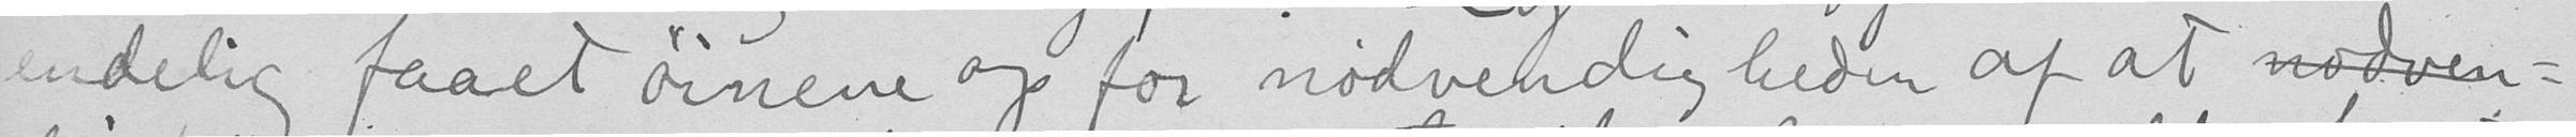endelig faaet øinene op for nødvendigheden af at
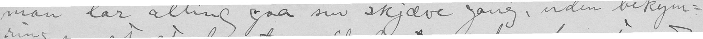man lar alting gaa sin skjæve gang, uden bekym-
